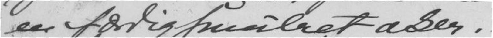en færdigsmulret aker.
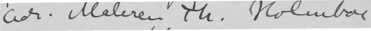Adr. Maleren Th. Holmboe
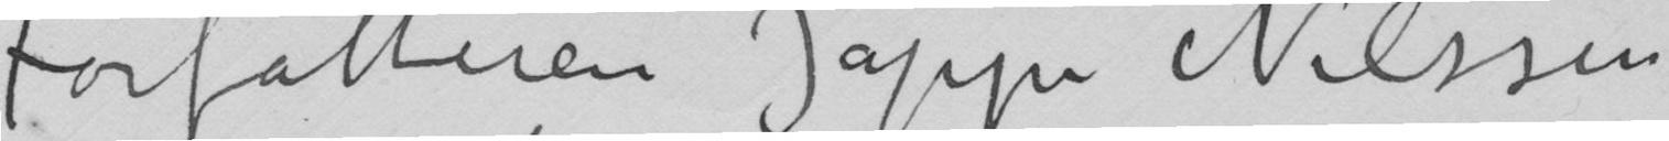Forfatteren Jappe Nilssen
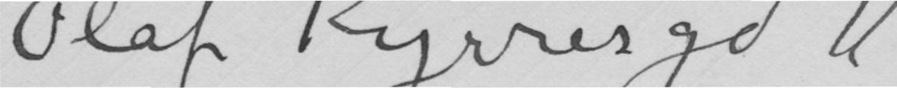Olaf Kyrresgd 11
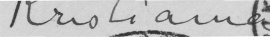Kristiania
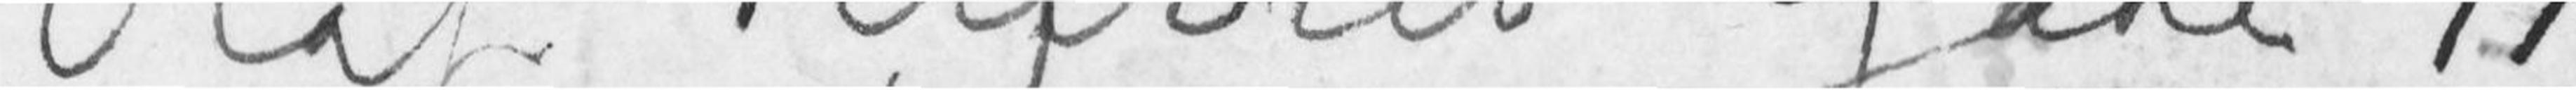Olaf Kyrres Gade 11
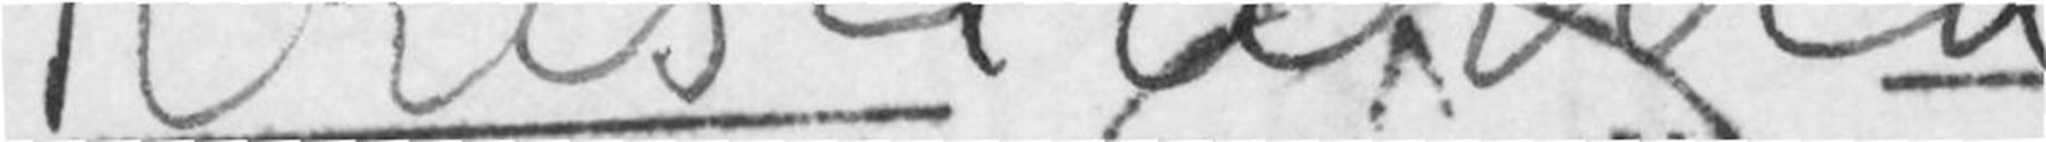Kristiania
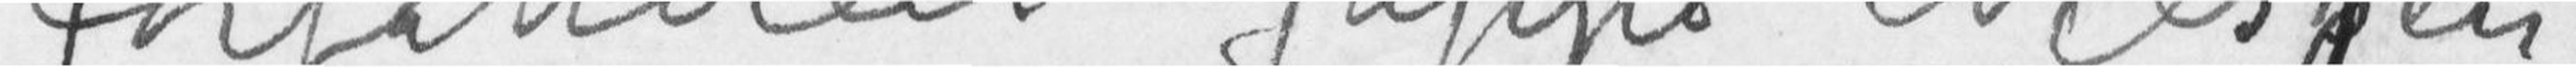Forfatteren Jappe Nilsssen
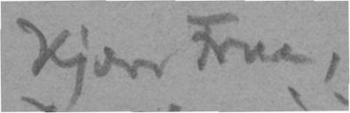Kjære Frue,
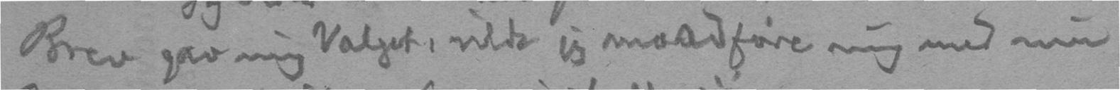Brev gav mig Valget, vilde jeg raadføre mig med min
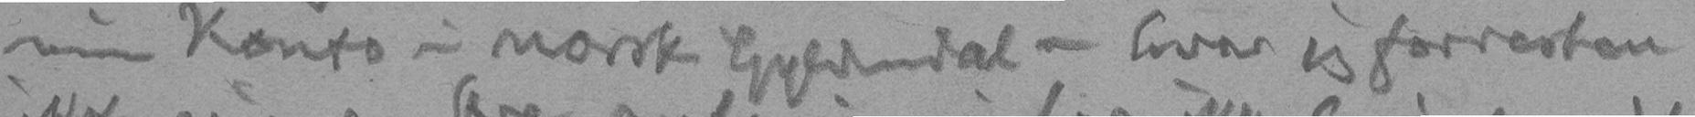min Konto i norsk Gyldendal - hvor jeg forresten
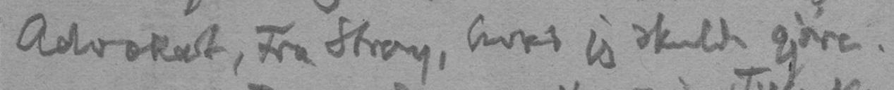Advokat, Fru Stray, hvad jeg skulde gjøre.
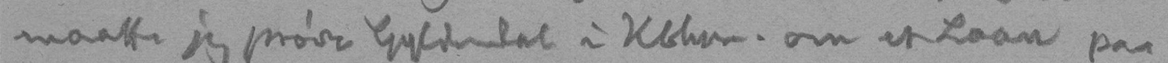maatte jeg prøve Gyldendal i Kbhvn. om et Laan paa
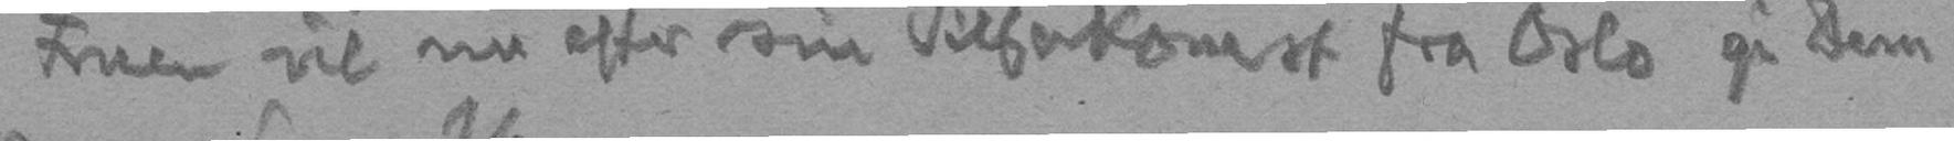Fruen vil nu efter sin Tilbakekomst fra Oslo gi Dem
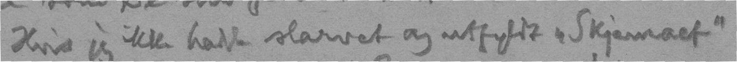Hvis jeg ikke hadde slarvet og utfyldt "Skjemaet"
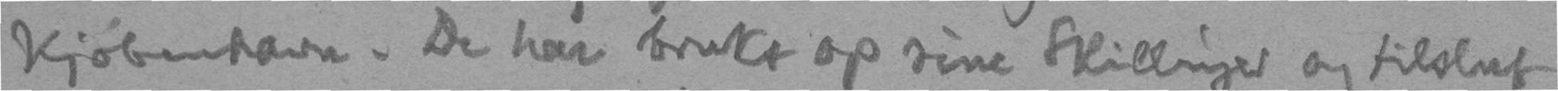Kjøbenhavn. De har brukt op sine Skillinger og tilslut
